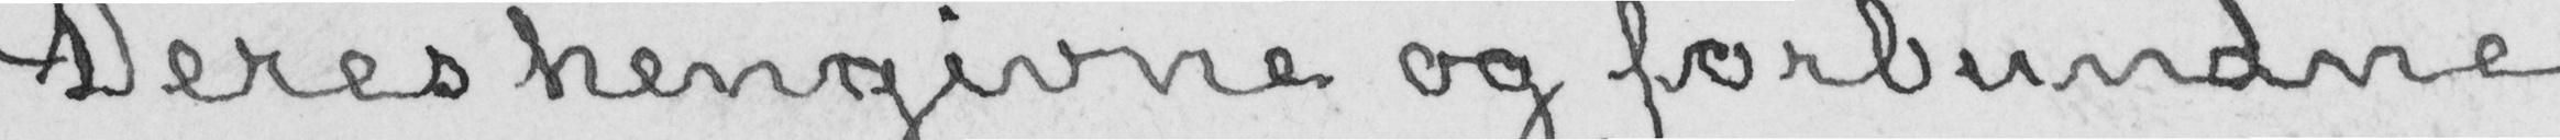Deres hengivne og forbundne
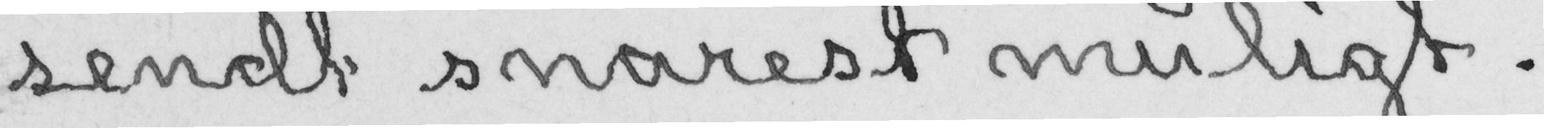sendt snarest muligt.
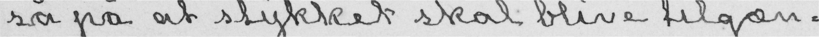så på at stykket skal blive tilgæn-
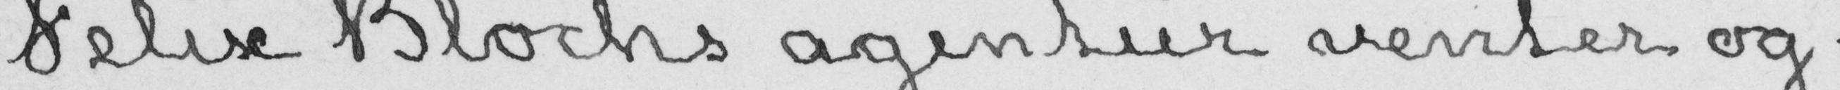Felix Blochs agentur venter og-
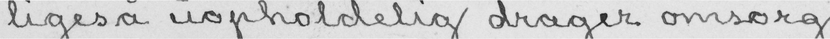ligeså uopholdelig drager omsorg
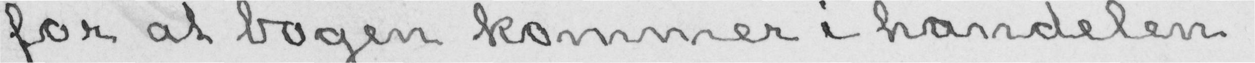for at bogen kommer i handelen.
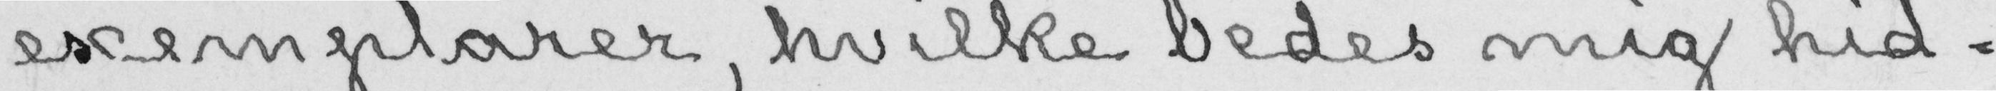exemplarer, hvilke bedes mig hid-
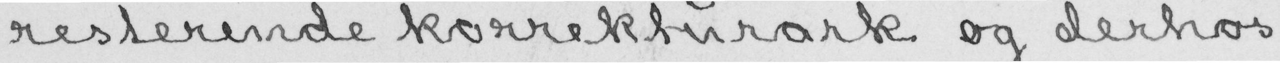resterende korrekturark og derhos
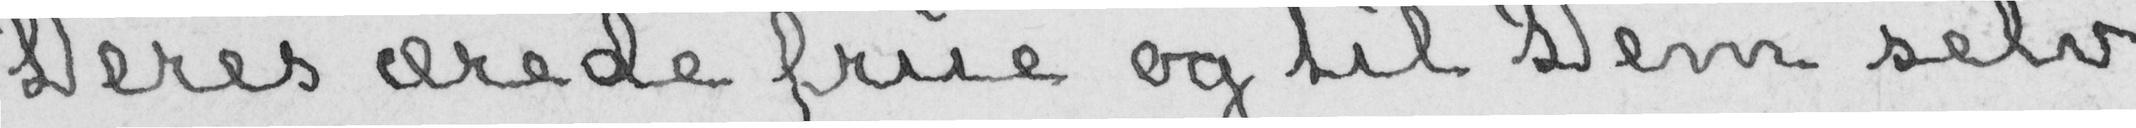Deres ærede frue og til Dem selv.
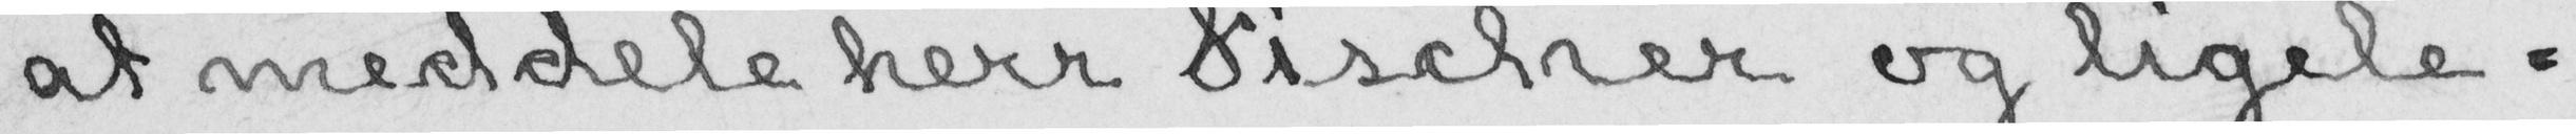at meddele herr Fischer og ligele-
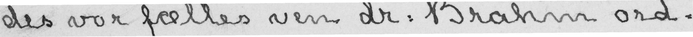des vor fælles ven dr: Brahm ord-
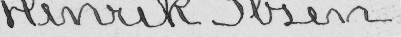Henrik Ibsen.
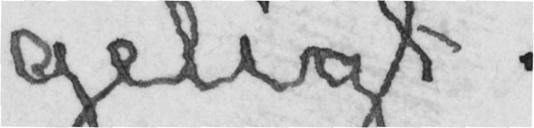geligt.
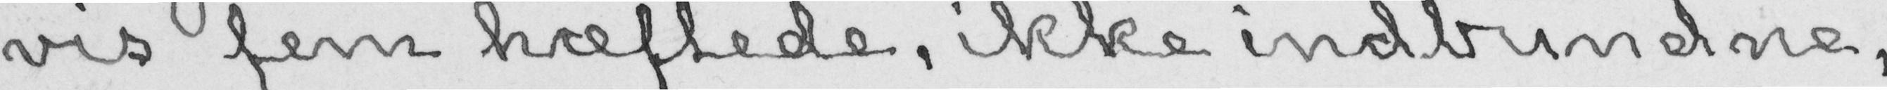vis fem hæftede, ikke indbundne,
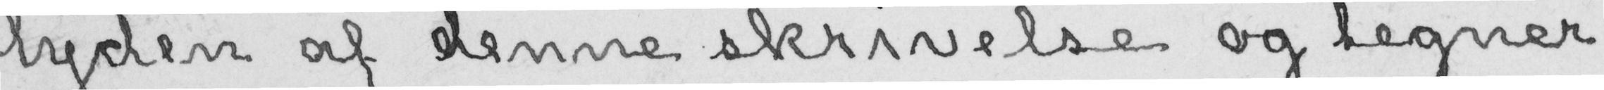lyden af denne skrivelse og tegner
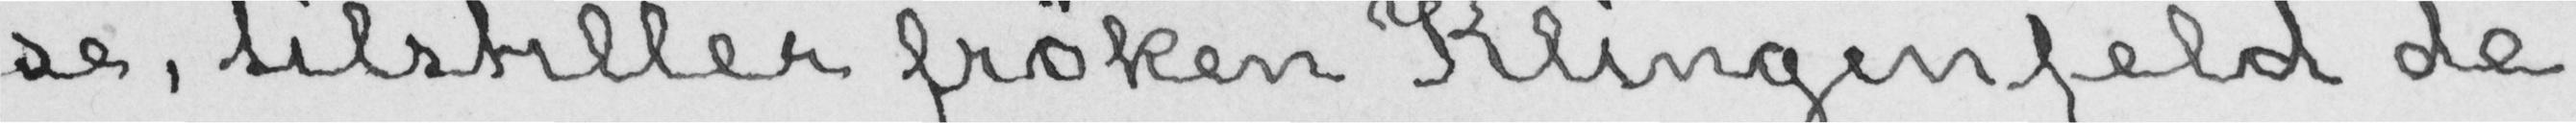se, tilstiller frøken Klingenfeld de
